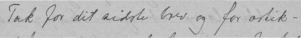Tak for dit sidste brev og for artik-
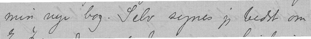min nye bog. Selv synes jeg bedst om
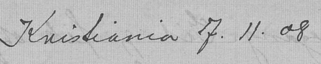Kristiania 7.11.08
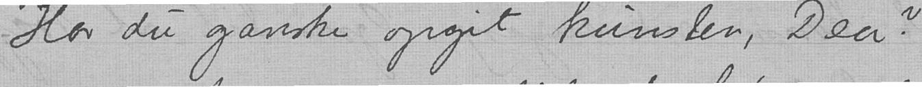Har du ganske opgit kunsten, Dea?
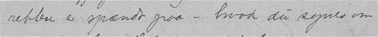rettere er spændt paa - hvad du synes om
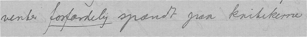venter forfærdelig spændt paa kritikerne
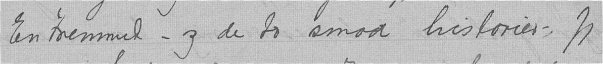En fremmed - og de to smaa historier. Jeg
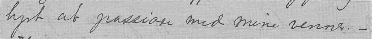lyst at passiare med mine venner. -
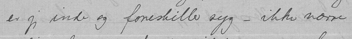er jeg inde og forestiller syg - ikke værre
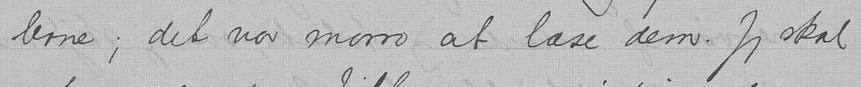lerne; det var moro at læse dem. Jeg skal
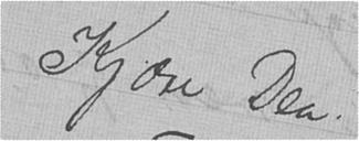Kjære Dea!
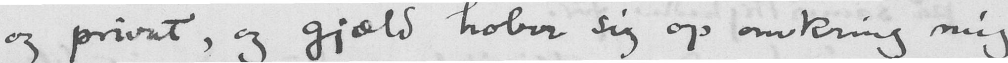og privat, og gjæld hober sig op omkring mig
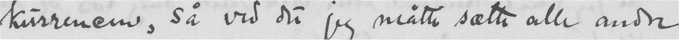kurrencen, så ved det jeg måtte sætte alle andre
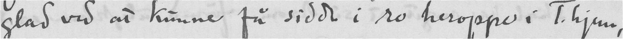glad ved at kunne få sidde i ro heroppe i T.hjem,
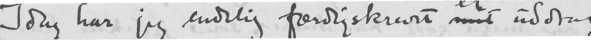Idag har jeg endelig færdigskrevet et uddrag
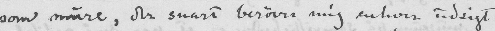som mure, der snart berøver mig enhver udsigt.
